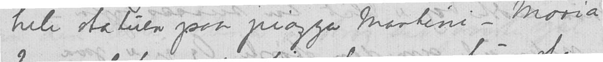hele statuen paa piazza Martiri - Maria
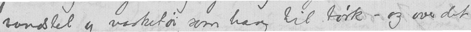vandstel og vasketøi som hang til tørk - og over det
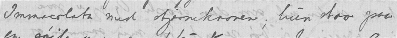Immacolata med stjernekronen; hun staar paa
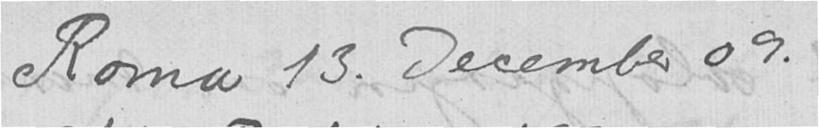Roma 13. December 09.
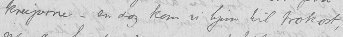kneiperne - en dag kom vi hjem til frokost,
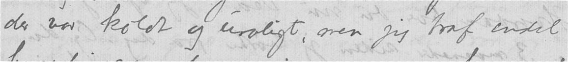der var koldt og uroligt, men jeg traf endel
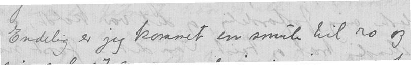Endelig er jeg kommet en smule til ro og
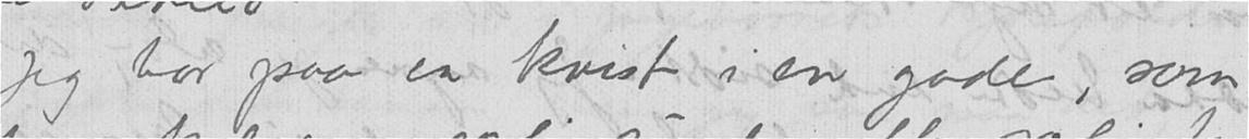Jeg bor paa en kvist i en gade, som
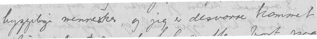hyggelige mennesker, og jeg er desværre kommet
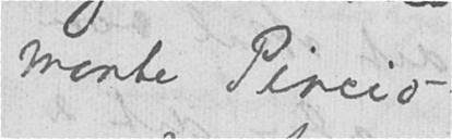Monte Pincio.
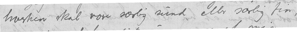hverken skal være særlig sund eller særlig fin,
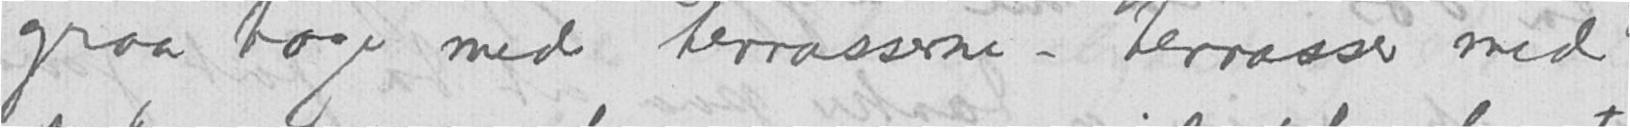graa tage med terrasserne - terrasser med
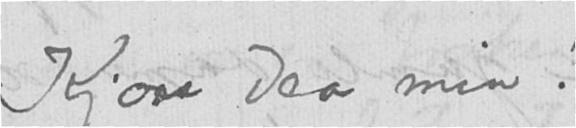Kjære Dea min!
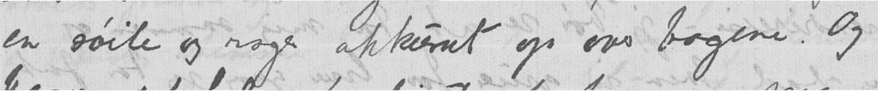en søile og rager akkurat op over tagene. Og
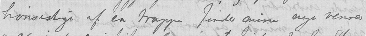min hønsestige af en trappe finder mine nye venner
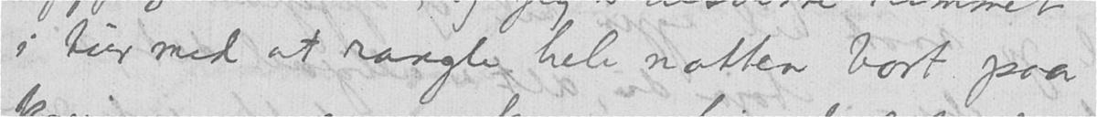i tur med at rangle hele natten bort paa
